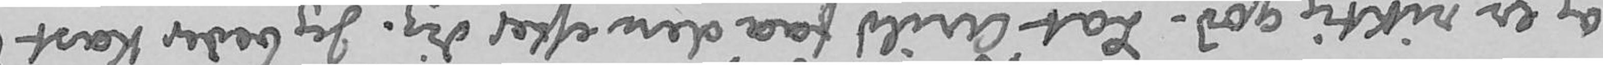og er riktig god. Lat Arild faa den efter dig. Jeg beder kast
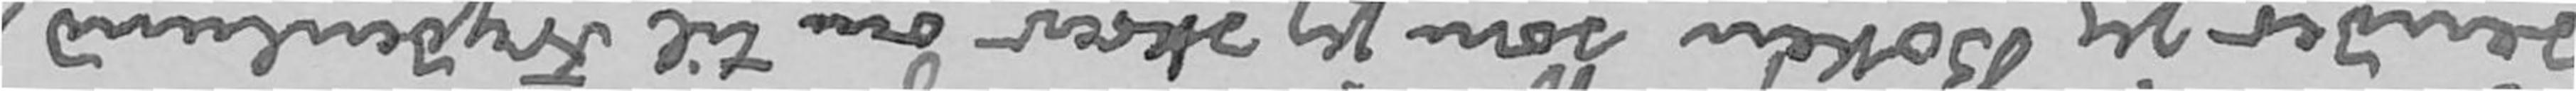sender jeg Boken som jeg skrev om til Frydenlund,
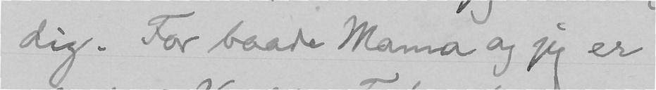dig. For baade Mama og jeg er
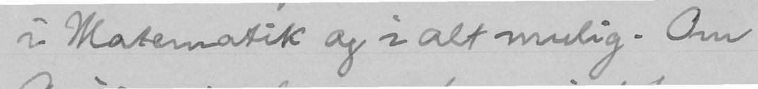i Matematik og i alt mulig. Om
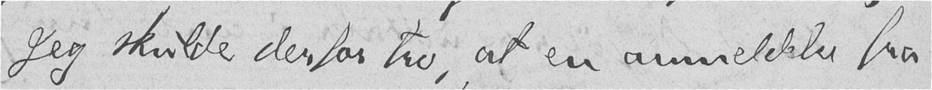Jeg skulde derfor tro, at en anmeldelse fra
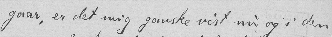gaar, er det mig ganske vist nu og i den
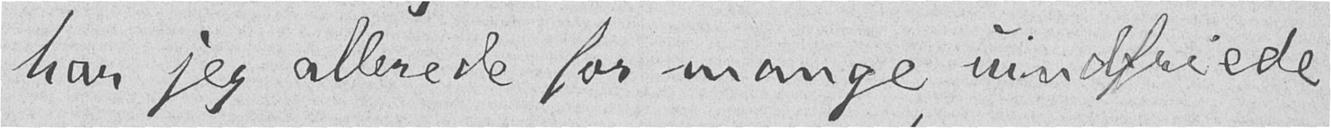har jeg allerede for mange uindfriede
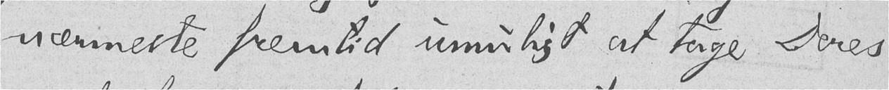nærmeste fremtid umuligt at tage Deres
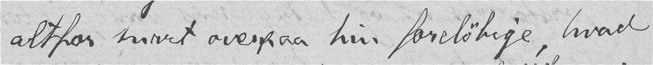altfor snart overpaa hin foreløbige, hvad
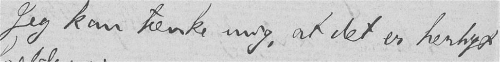Jeg kan tænke mig, at det er herligt
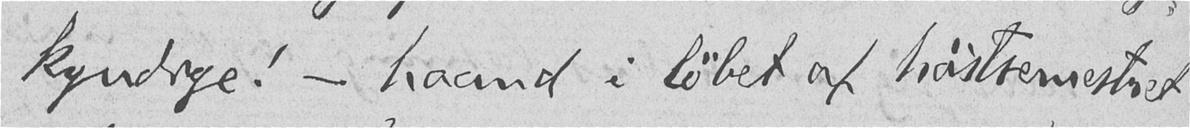kyndige! - haand i løbet af høstsemestret
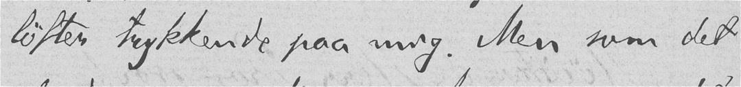løfter trykkende paa mig. Men som det
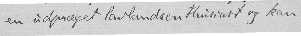en udpræget lavlandsenthusiast og kan
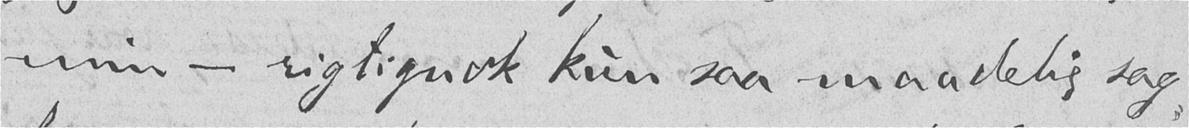min - rigtignok kun saa maadelig sag-
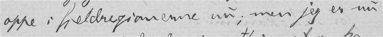oppe i fjeldregionerne nu; men jeg er nu
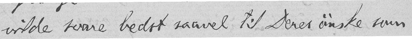vilde svare bedst saavel til Deres ønske som
